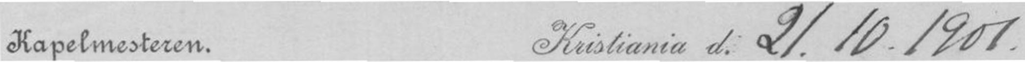Kapelmesteren.Kristiania d. 21.10.1901
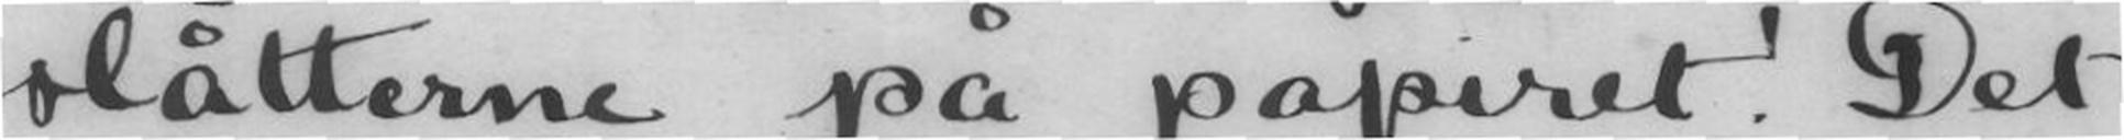slåtterne på papiret! Det
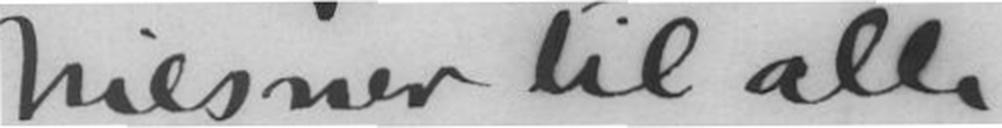hilsener til alle
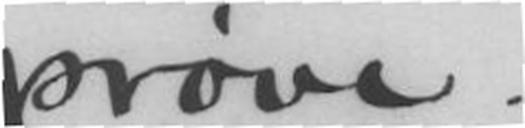prøve.
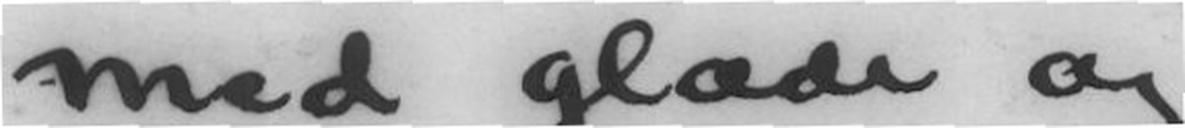med glæde og
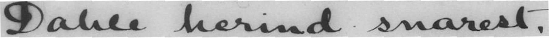Dahle herind snarest,
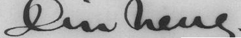Din heng.
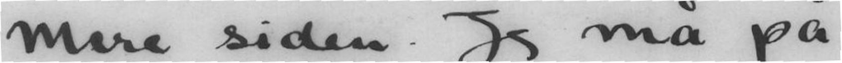Mere siden. Jeg må på
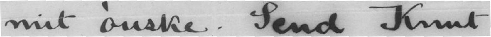mit ønske. Send Knut
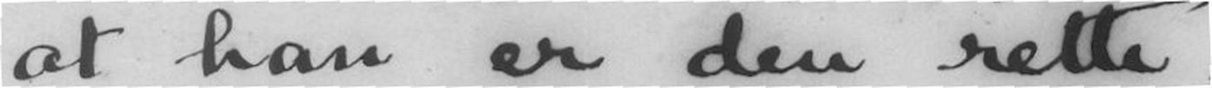at han er den rette.
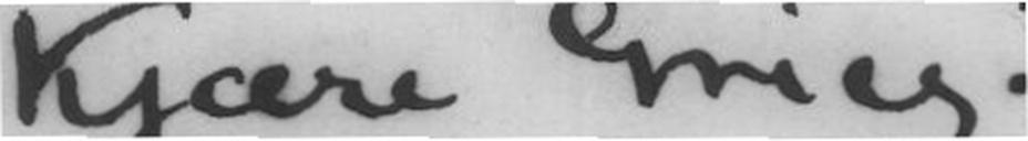Kjære Grieg!
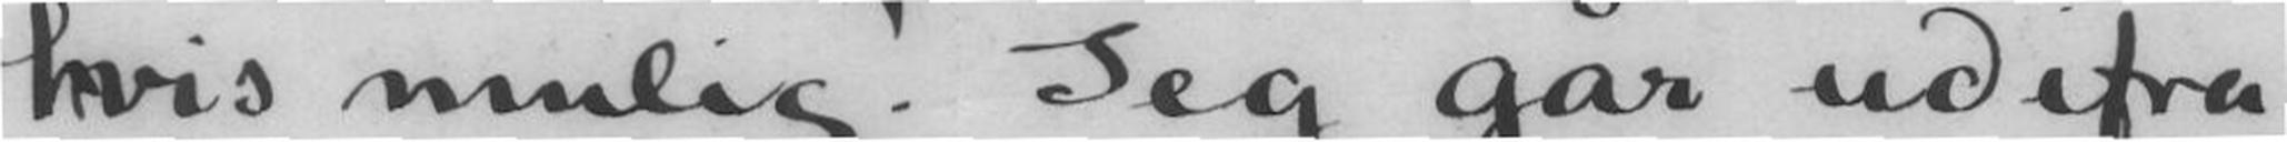hvis mulig! Jeg går udifra
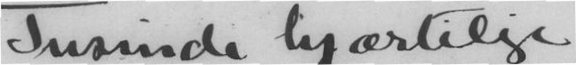Tusinde hjærtelige
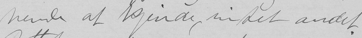hende at kjende, intet andet.
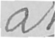at
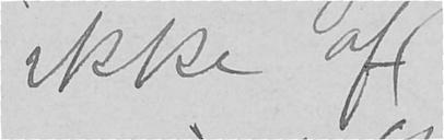ikke af
